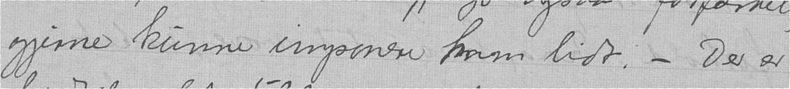gjerne kunne imponere ham lidt. — Der er
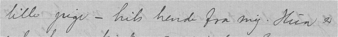lille pige — hils hende fra mig. Hun er
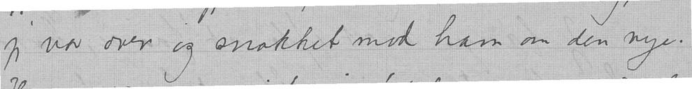jeg var over og snakket med ham om den nye.
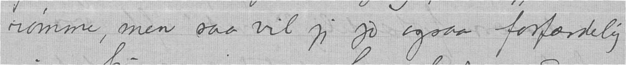rømme, men saa vil jeg jo ogsaa forfærdelig
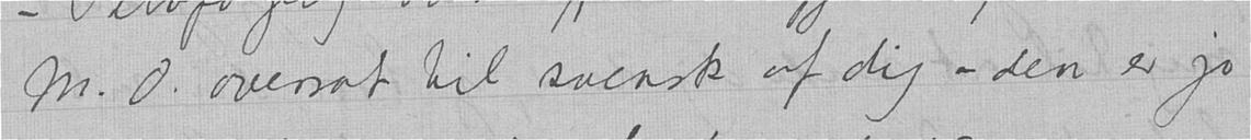M. O. oversat til svensk af dig — den er jo
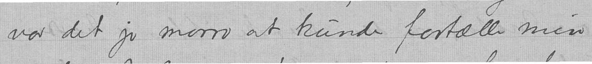var det jo morro at kunde fortælle min
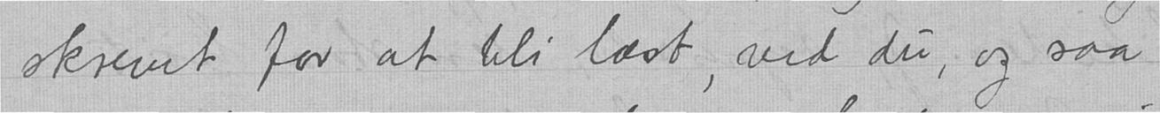skrevet for at bli læst, ved du, og saa
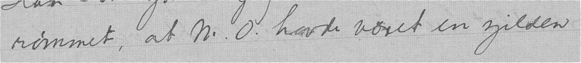rømmet, at M. O. havde været en sjelden
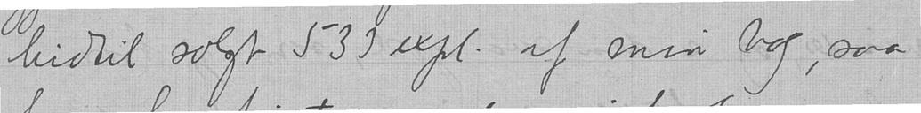hidtil solgt 531 expl. af min bog, saa
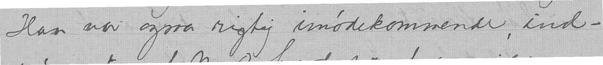Han var ogsaa rigtig imødekommende, ind-
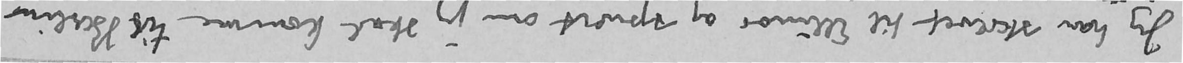Jeg har skrevet til Ellinor og spurt om jeg skal komme til Berlin
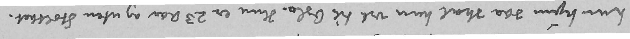hun hjem saa skal hun vel til Oslo. Hun er 23 Aar og uten Stolthet.
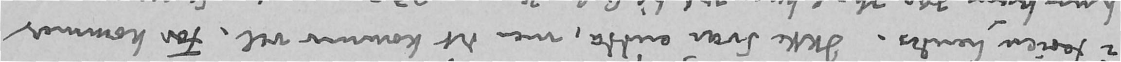i Ferien hendes. Ikke Svar endda, men det kommer vel. For kommer
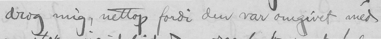drog mig, nettop fordi den var omgivet med
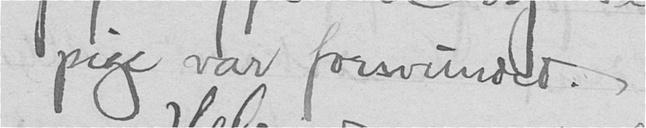pige var forsvundet.
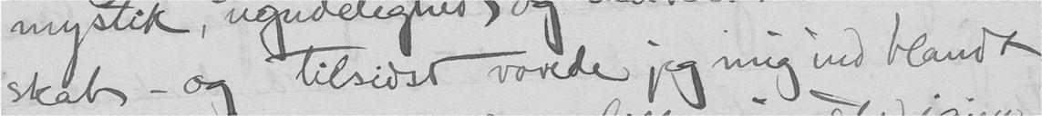skab - og tilsidst vovde jeg mig ind blandt
